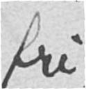fri
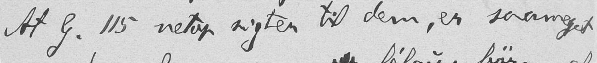At G. 115 netop sigter til dem, er saameget
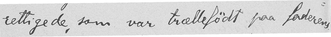rettigede, som var trællefødt paa faderens
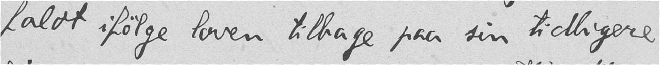faldt ifølge loven tilbage paa sin tidligere
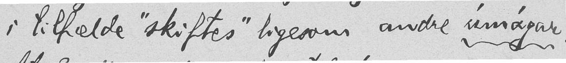i tilfælde "skiftes" ligesom andre úmágar.
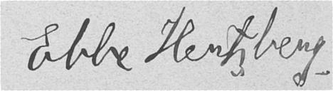Ebbe Hertzberg.
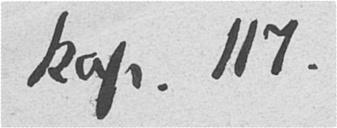kap. 117.
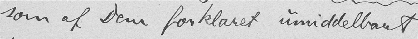som af Dem forklaret umiddelbart
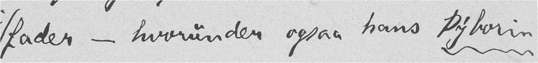fader - hvorunder ogsaa hans Þýborin
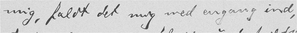mig, faldt det mig med engang ind,
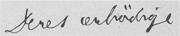Deres ærbødige
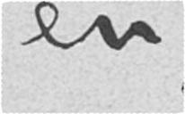en
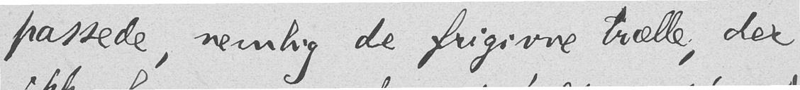passede, nemlig de frigivne trælle, der
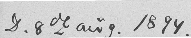D. 8de aug. 1894.
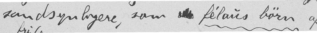sandsynligere, som  félaus børn af
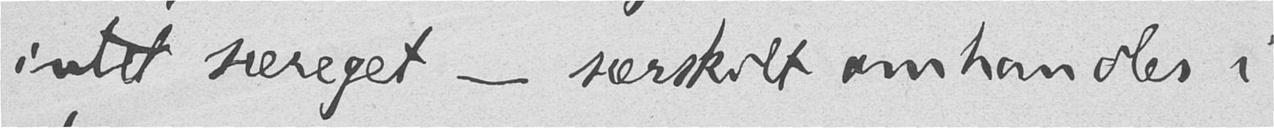intet særeget - særskilt omhandles i
